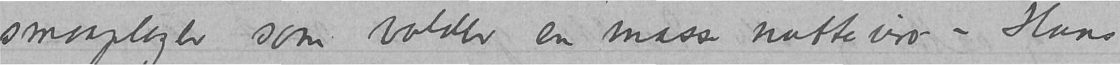smaaplager som volder en masse natteuro – Hans
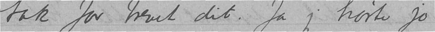tak for brevet dit. Ja jeg hørte jo
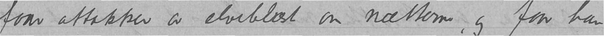faar attakker av elveblæst om nætterne, og faar han
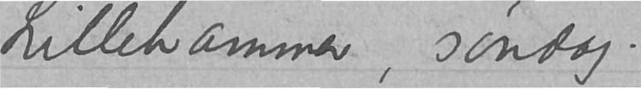Lillehammer, søndag
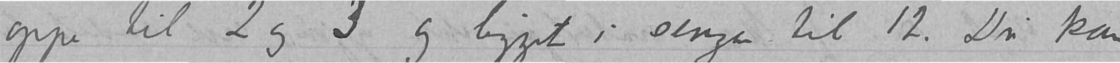oppe til 2 og 3 og ligget i sengen til 12. Du kan
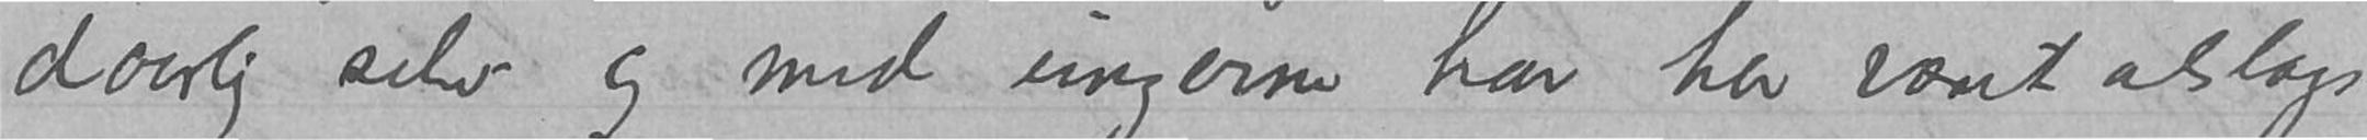daarlig selv og med ungerne har her været alslags
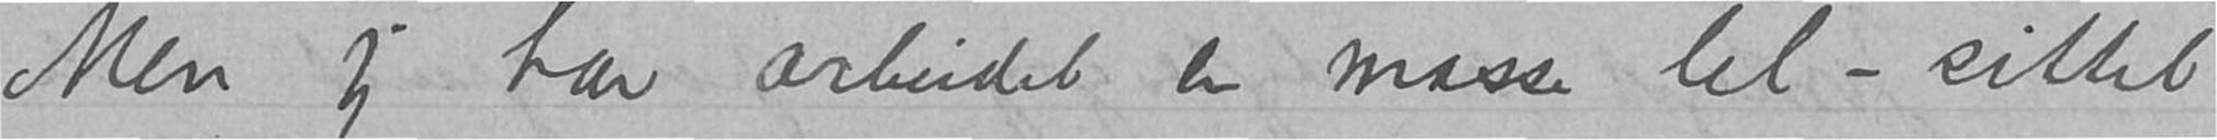Men jeg har arbeidet en masse lel – sittet
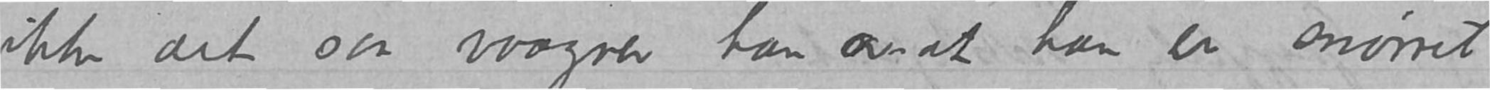ikke det saa vaagner han av at han er snørret
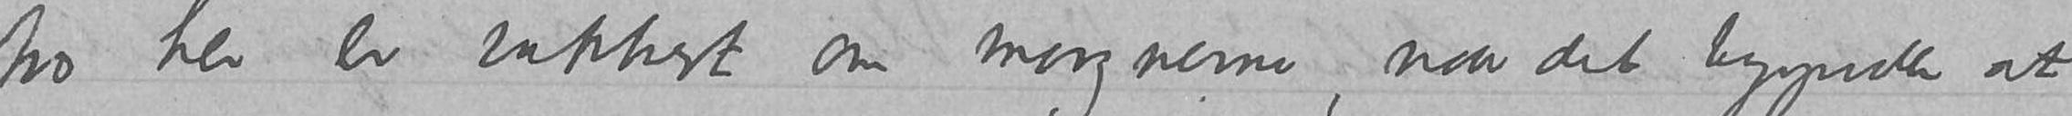tro her er vakkert om morgnerne, naar det begynder at
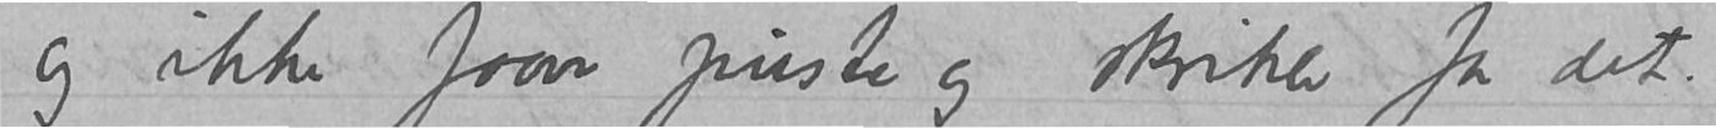og ikke faar puste og skriker for det.
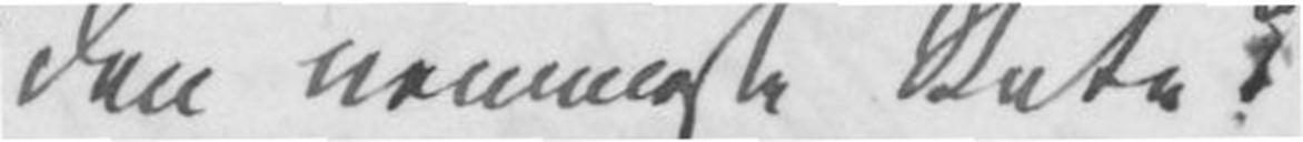den nemmeste Rute?
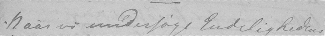Naar vi undersøge Endelighedens
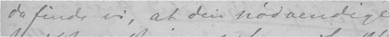da finde vi, at den nødvendige
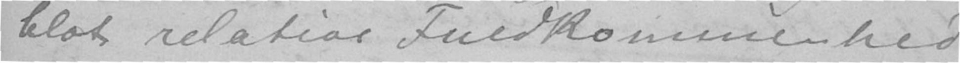blot relative Fuldkommenhed,
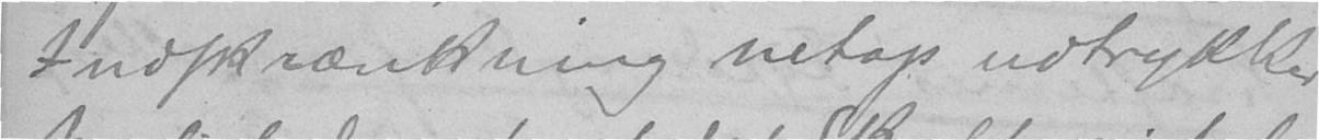Indskrænkning netop udtrykker
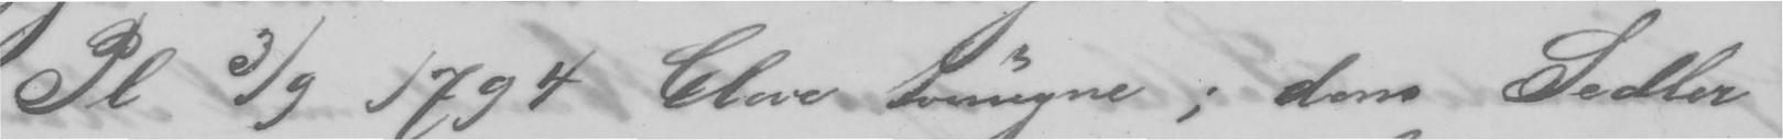Pl 3/9 1794 bleve tvungne; dens Sedler
